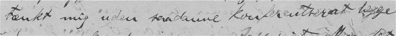tænkt mig uden saadanne konferentser at lægge
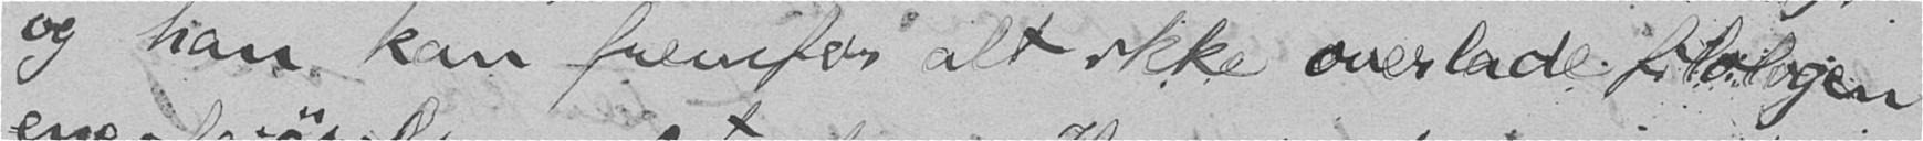og han kan fremfor alt ikke overlade filologen
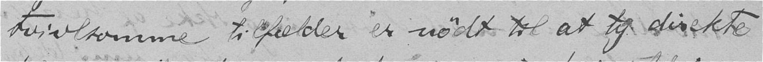tvivlsomme tilfælder er nødt til at ty direkte
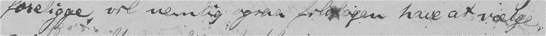foreligge, vil nemlig ogsaa filologen have at vælge
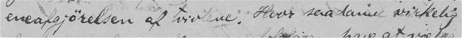eneafgjørelsen af tvivlene. Hvor saadanne virkelig
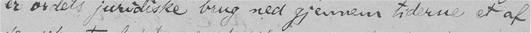er ordets juridiske brug ned gjennem tiderne et af
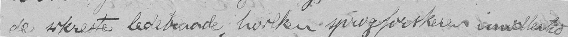de sikreste ledetraade, hvilken sprogforskeren imidlertid
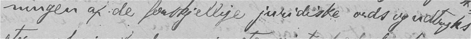ningen af de forskjellige juridiske ords og udtryks
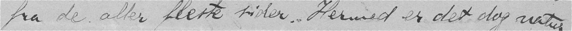fra de aller fleste sider. Hermed er det dog natur-
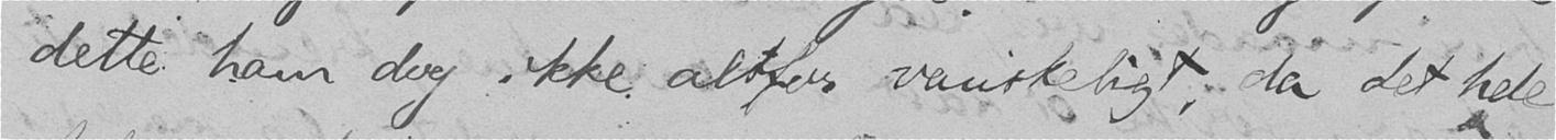dette ham dog ikke altfor vanskeligt, da det hele
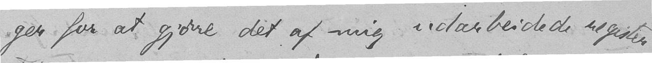ger for at gjøre det af mig udarbeidede register
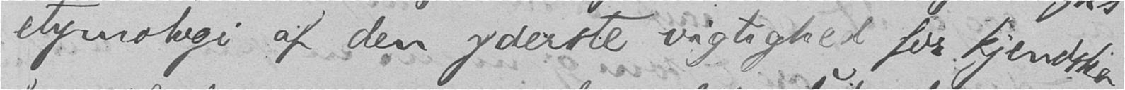etymologi af den yderste vigtighed for kjendska-
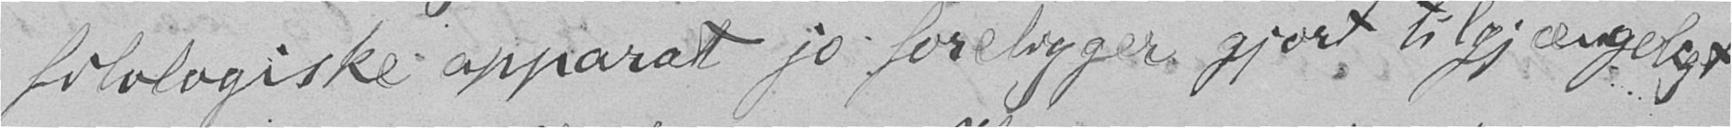filologiske apparat jo foreligger gjort tilgjængeligt
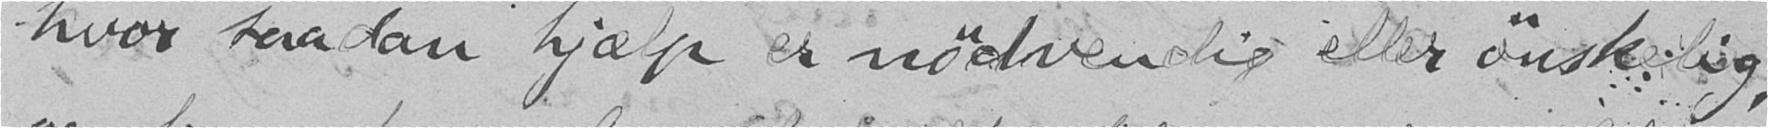hvor saadan hjælp er nødvendig eller ønskelig,
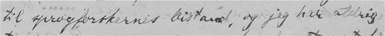til sprogforskernes bistand, og jeg har aldrig
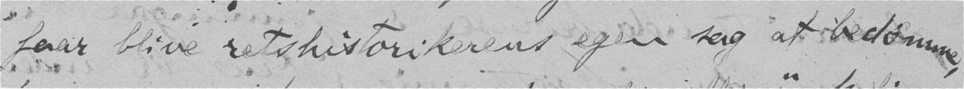faar blive retshistorikerens egen sag at bedømme,
